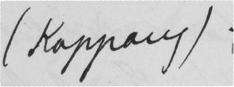(Koppang).
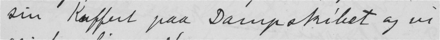sin Koffert paa Dampskibet og vi
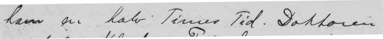ham en halv Times Tid. Doktoren
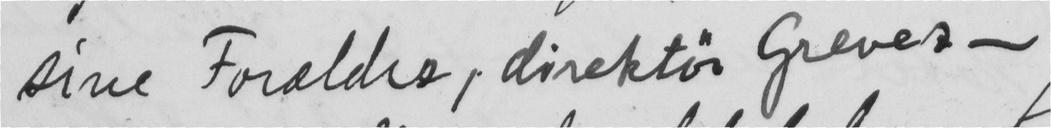sine Forældre, direktør Grever –
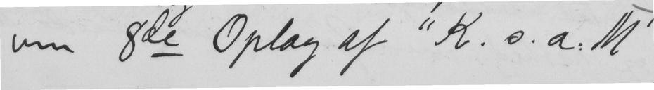om 8de Oplag af "K. s. a. M."
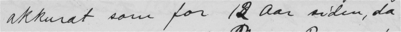akkurat som for 12 Aar siden, da
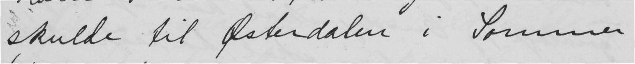skulde til Østerdalen i Sommer
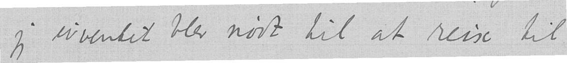jeg uventet blev nødt til at reise til
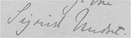Sigrid Undset.
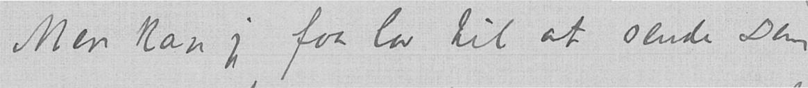Men kan jeg faa lov til at sende Dem
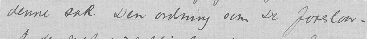denne sak. Den ordning som De foreslaar –
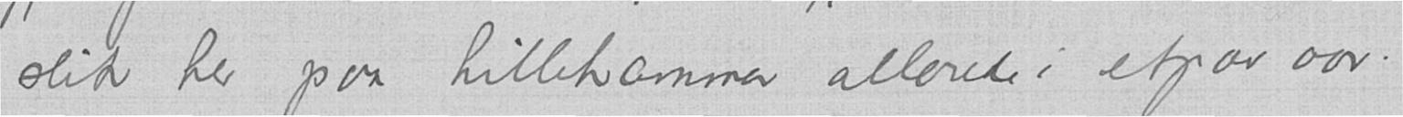slik her paa Lillehammer allerede i etpar aar.
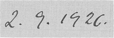2.9.1926.
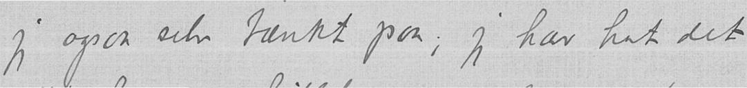jeg ogsaa selv tænkt paa; jeg har hat det
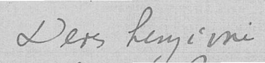Deres hengivne
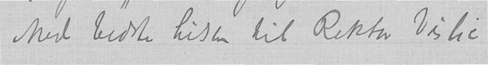Med bedste hilsen til Rektor Vislie
